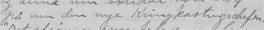på um den nye Kringkastingschefen.
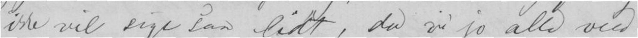ikke vil sige saa lidt, da vi jo alle veed
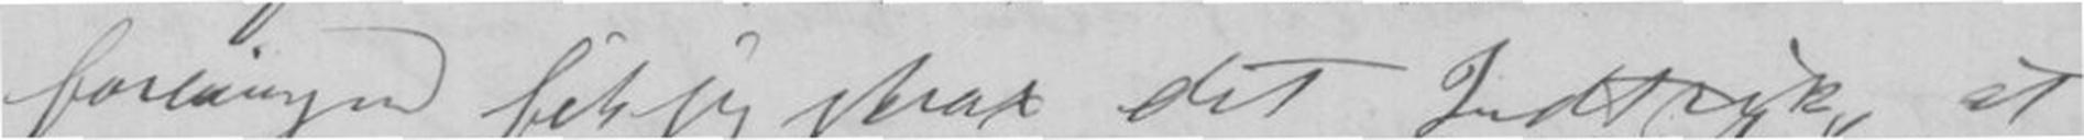foreningen fik jeg strax det Indtryk, at
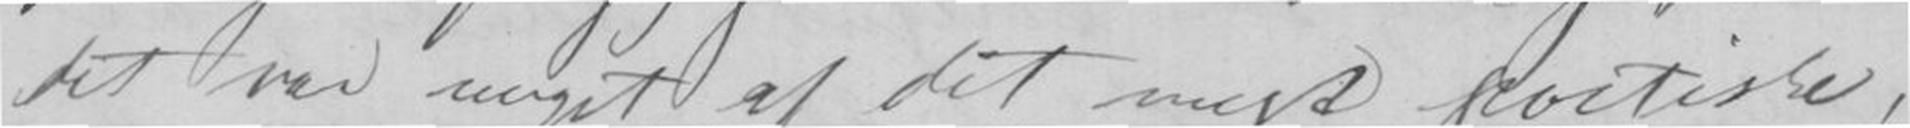det var meget af det mest poetiske,
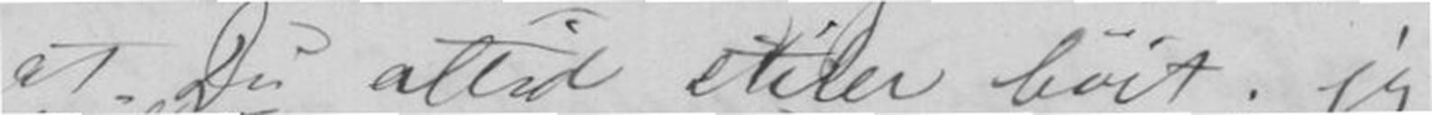at Du altid stiler høit; jeg
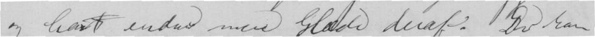og havt endnu mere Glæde deraf. Du kan
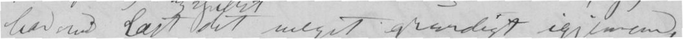har nu læst det meget grundigt igjennem,
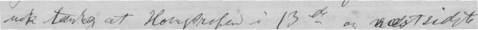nok tænke, at Hornstrofen i 13de og næst sidste
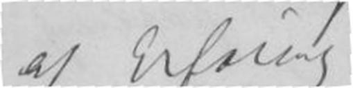af Erfaring,
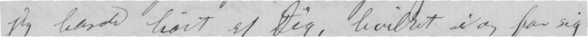jeg havde hørt af Dig, hvilket i og for sig
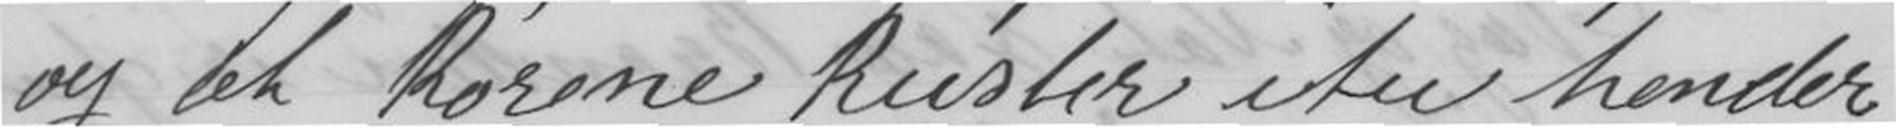og at Rørene Ruster itu hender
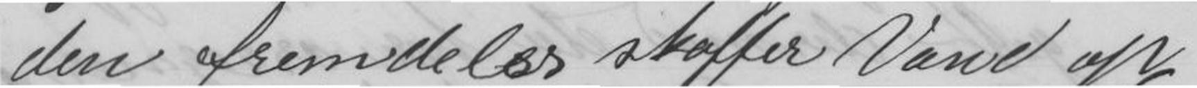den fremdeles skaffer Vand op,
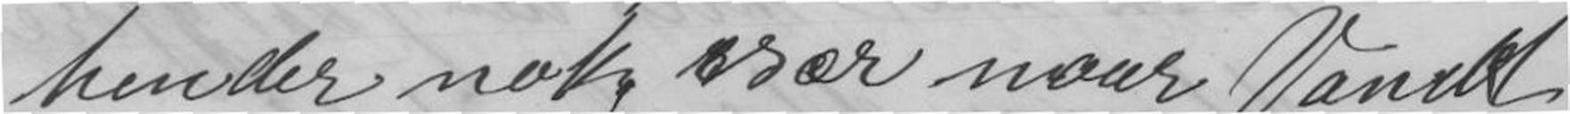hender nok, især naar Vand
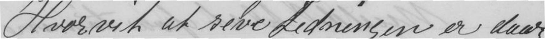Hvorvit at selve Ledningen er daar-
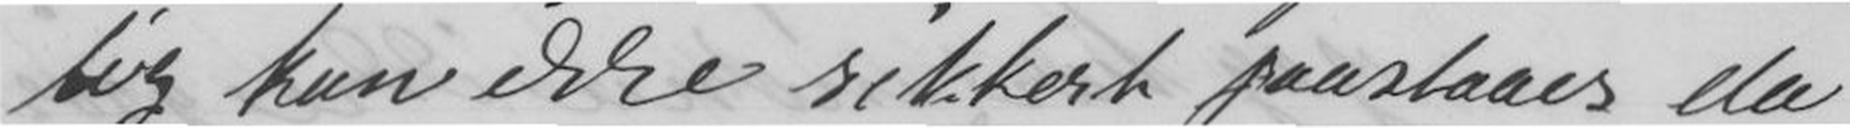lig kan ikke sikkert paastaaes da
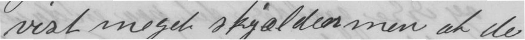vist meget skjælden men at de
